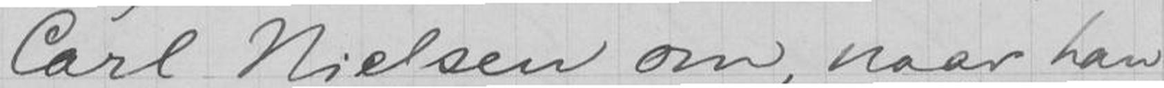Carl Nielsen om, naar han
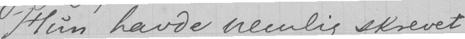Hun havde nemlig skrevet
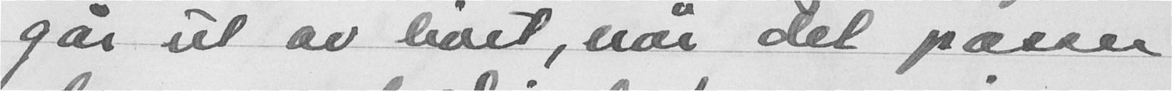går ut av livet, når det passer
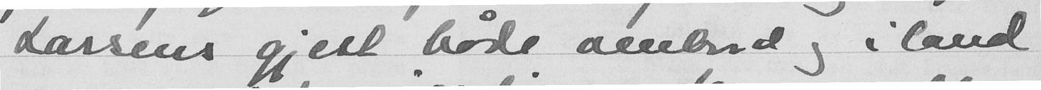Larsens gjest både ombord og i land
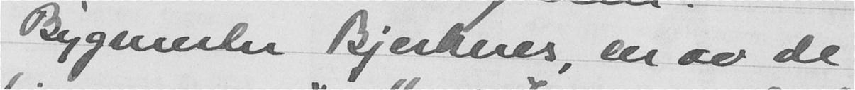Bygmester Bjerknes, en av de
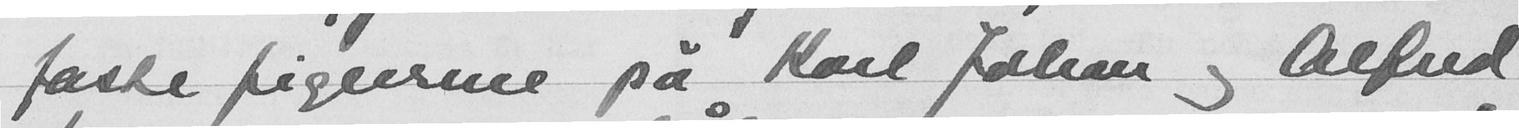faste figurene på Karl Johan og Alfred
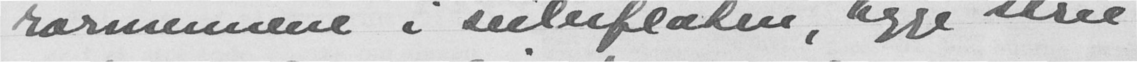rormennene i seilerflåten, begge strie
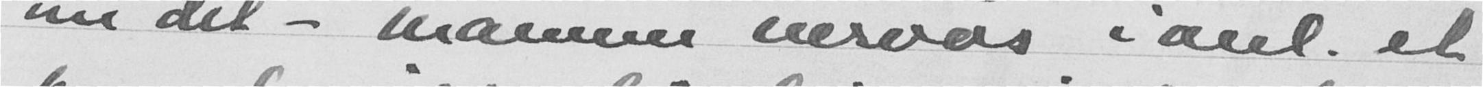om det - mannen nervøs i anl. et
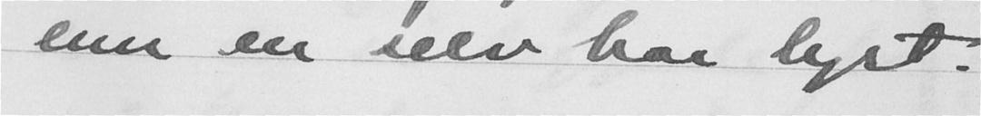enn en selv har lyst.
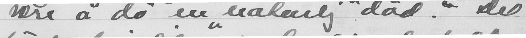være å dø en naturlig død." Det
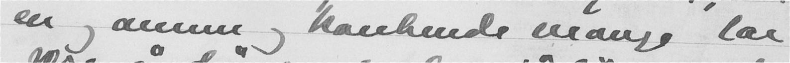en og annen og kanhende mange lar
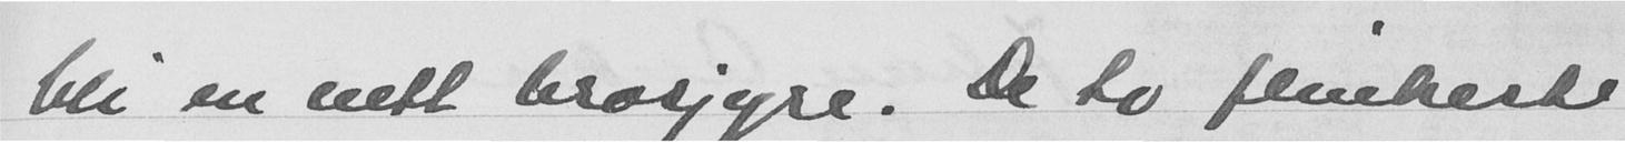bli en nett brosjyre. De to flinkeste
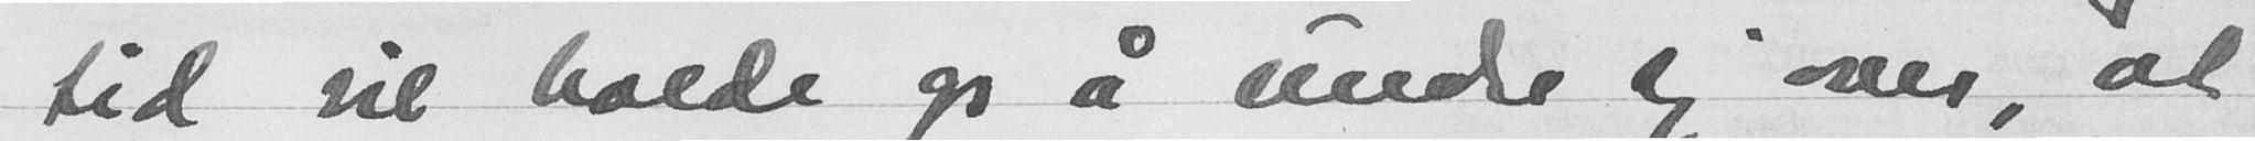tid vil holde op å undre sig over, at
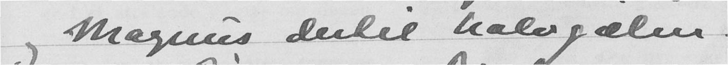og Magnus dertel halvgælen.
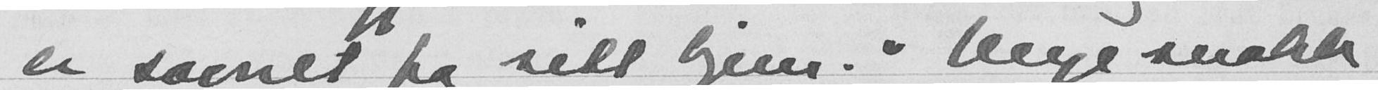er "savnet fra sitt hjem." Mye snakk
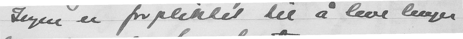Seyer er forpliktet til å leve lenger
